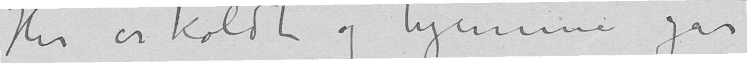Her er koldt og hjemme går
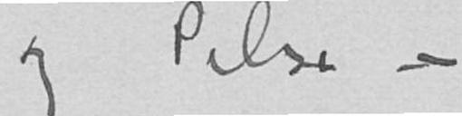og Pelse –
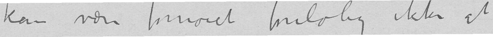kan være fornøiet foreløbig ikke at
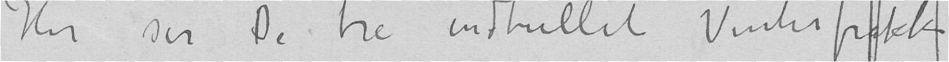Her ser Du tre indtullete Vinterfrakke
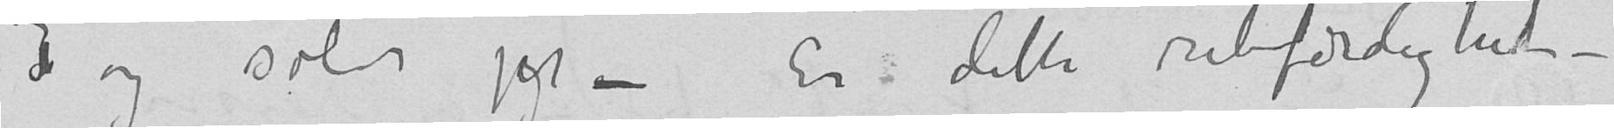I og soler jegr – Er dette retfærdighet –
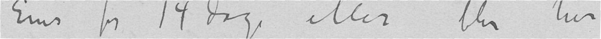Ems for 14 dage eller blir her
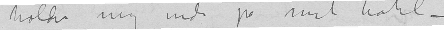holder mig inde på mit hotel –
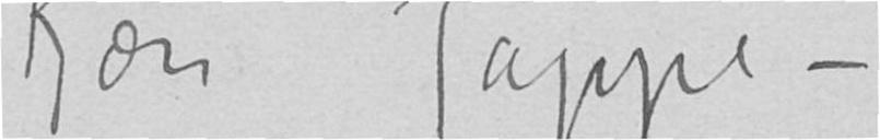Kjære Jappe –
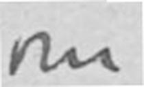nu
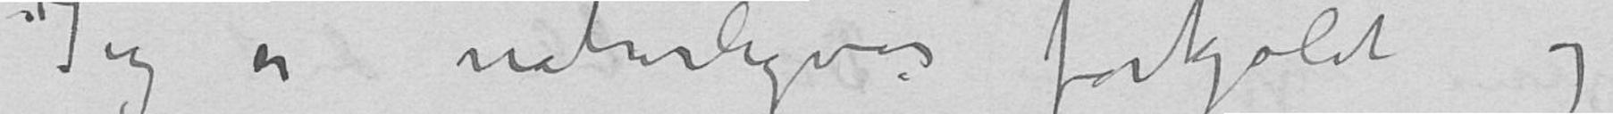Jeg er naturligvis forkjølet og
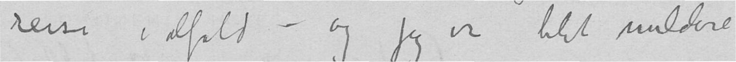reise ialfald – og jeg er blit mildere
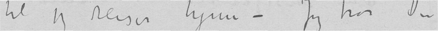til jeg reiser hjem – Jeg tror Du
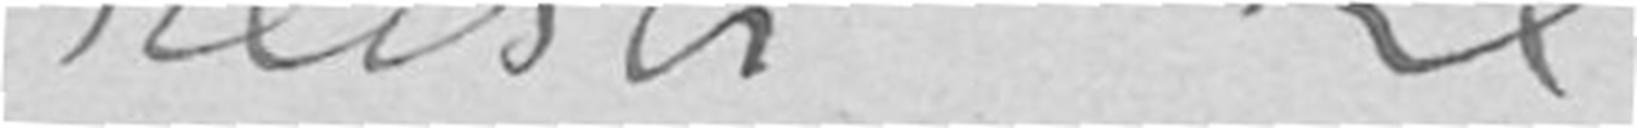reiser til
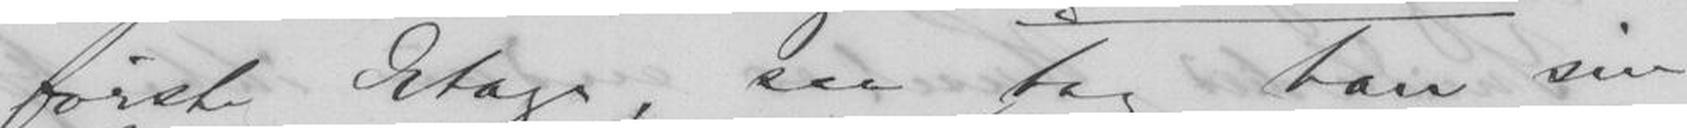første Etage, saa tog han sin
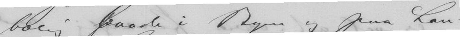bolig baade i Byen og paa Lan-
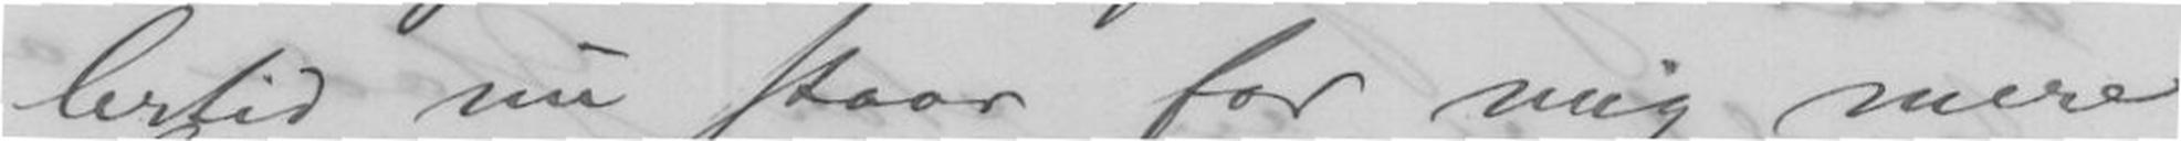lertid nu staar for mig mere
